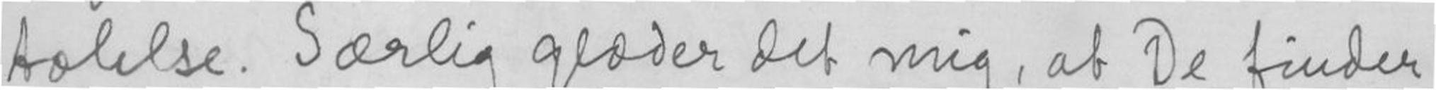talelse. Særlig glæder det mig, at De finder
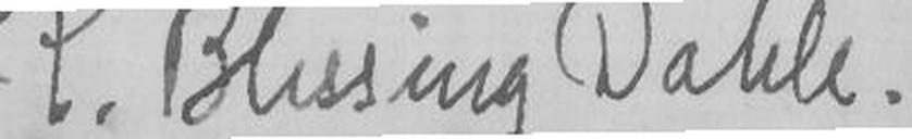P. Blessing Dahle.
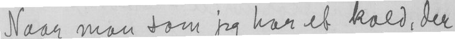Naar man som jeg har et kald, der
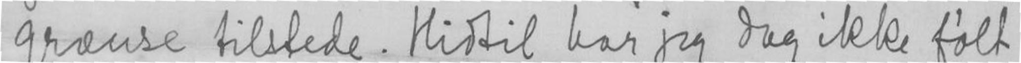grænse tilstede. Hidtil har jeg dog ikke følt
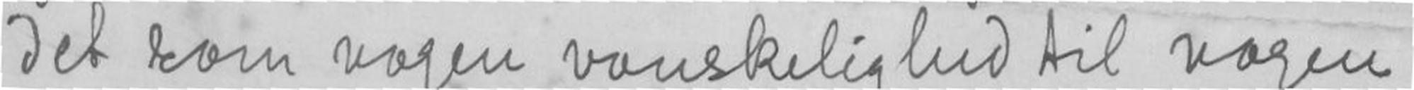det som nogen vanskelighed til nogen
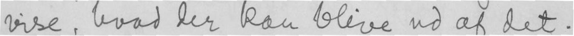vise, hvad der kan blive ud af det.
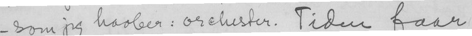- som jeg haaber i orchester. Tiden faar
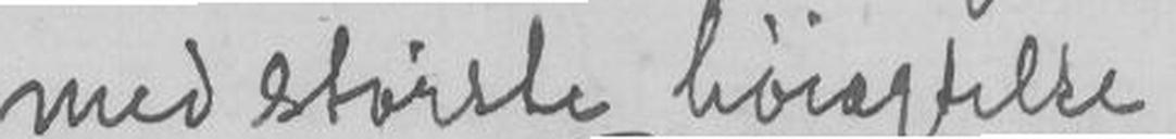med største høiagtelse
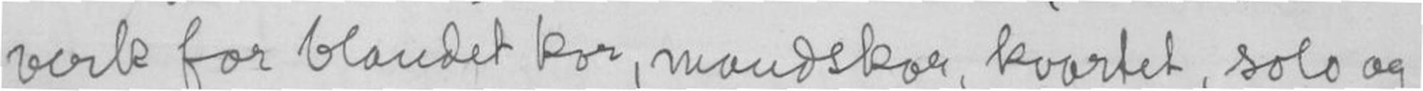verk for blandet kor, mandskor, kvartet, solo og
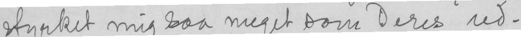styrket mig saa meget som Deres ud-
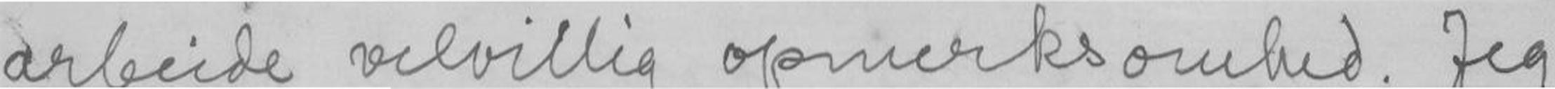arbeide velvillig opmerksomhed. Jeg
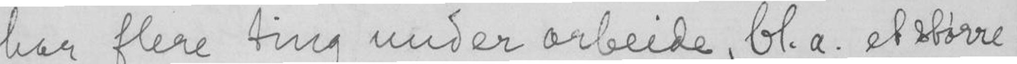har flere ting under arbeide, bl.a. et større
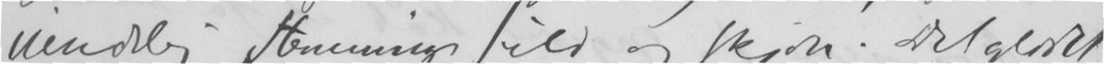uendelig stemnings fuld og skjødt. Det glædet
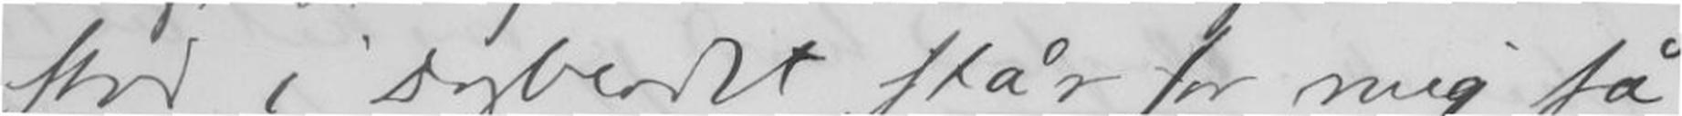stod i Dagbladet står for mig så
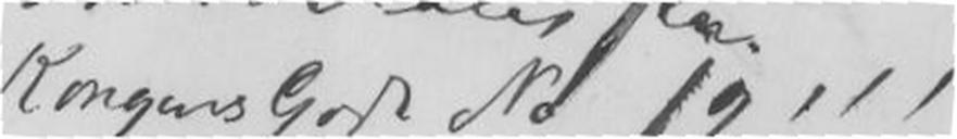Kongens Gade No 12!!!
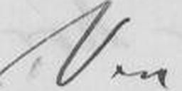Ven
